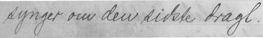synger om den sidste dragt.
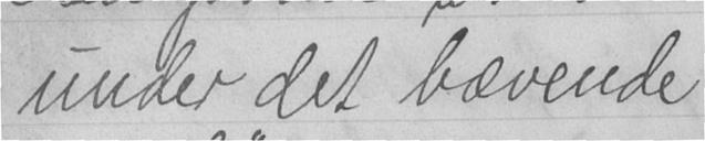under det bævende
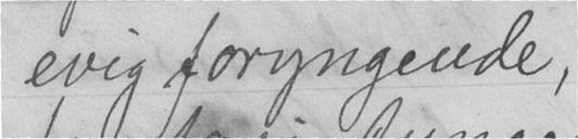evig foryngende,
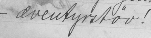- æventyrstøv!
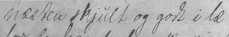næsten skjult og godt i læ
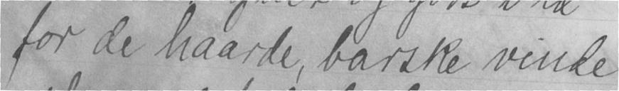for de haarde, barske vinde
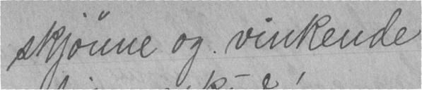skjønne og vinkende
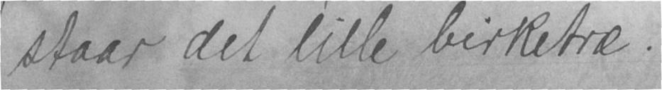staar det lille birketræ.
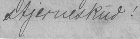stjerneskud!
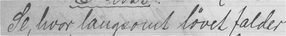Se, hvor langsomt løvet falder
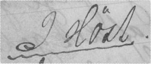I Høst.
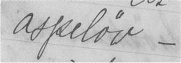aspeløv -
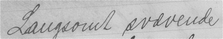Langsomt svævende
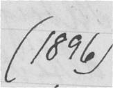(1896)
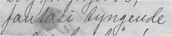fantasi syngende
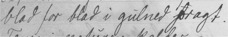blad for blad i gulned pragt
